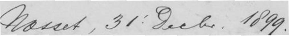Næsset, 31 Decb. 1899.
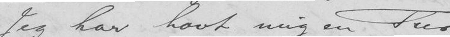Jeg har havt mig en Tur
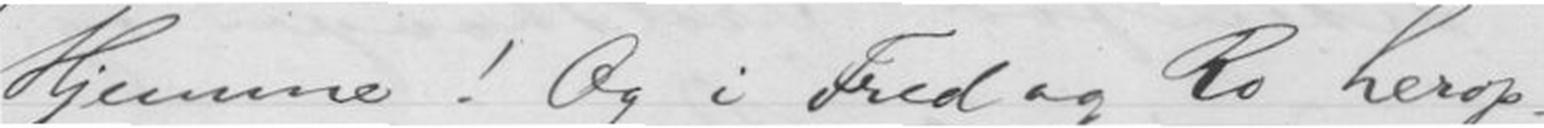Hjemme! Og i Fred og Ro herop-
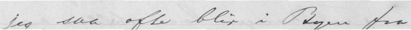jeg saa ofte blir i Byen fra
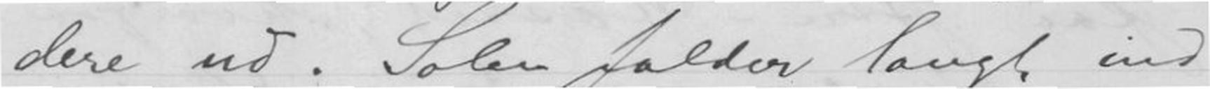dere ud. Solen falder langt ind
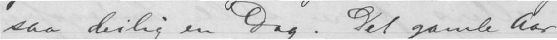saa deilig en Dag. Det gamle Aar
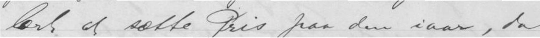lært at sætte Pris paa den iaar, da
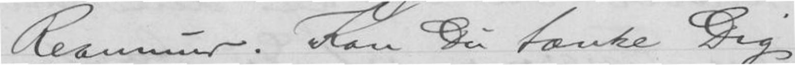Reaumur. Kan Du tænke Dig
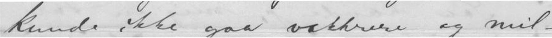kunde ikke gaa vakkrere og mil-
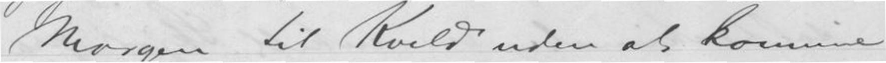Morgen til Kveld uden at komme
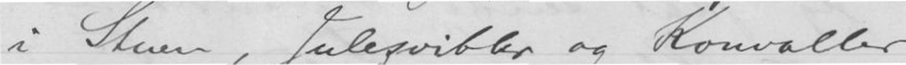i Stuen, Julezvibler og Konvaller
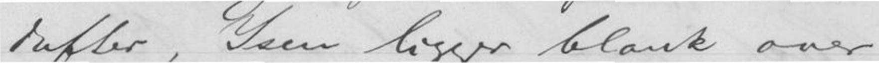dufter, Isen ligger blank over
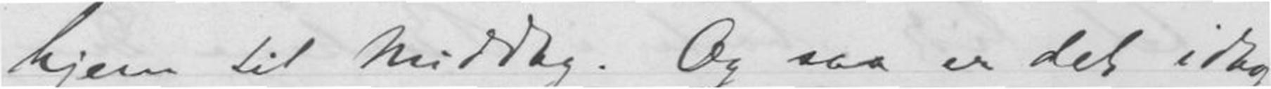hjem til Middag. Og saa er det idag
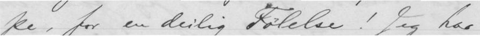pe, for en deilig Følelse! Jeg har
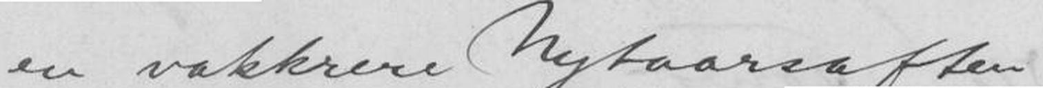en vakkrere Nytaarsaften
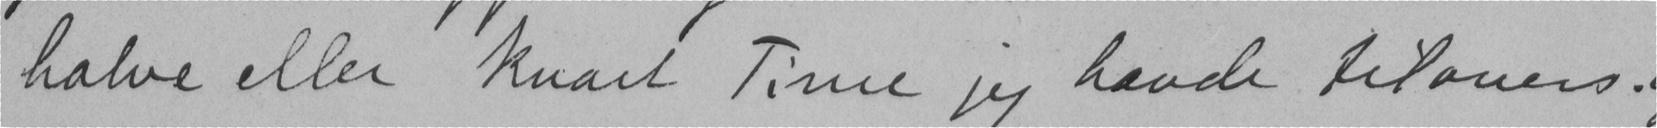halve eller kvart Time jeg havde tilovers.
